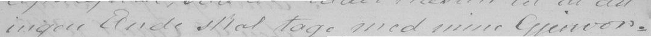ingen Ænde skal tage med mine Gjenvor-
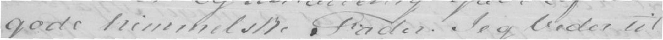gode himmelske Fader. Jeg beder til
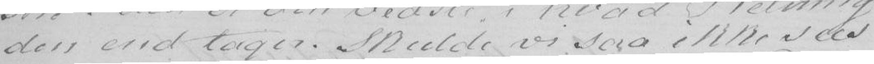den end tager. Skulde vi saa ikke sees
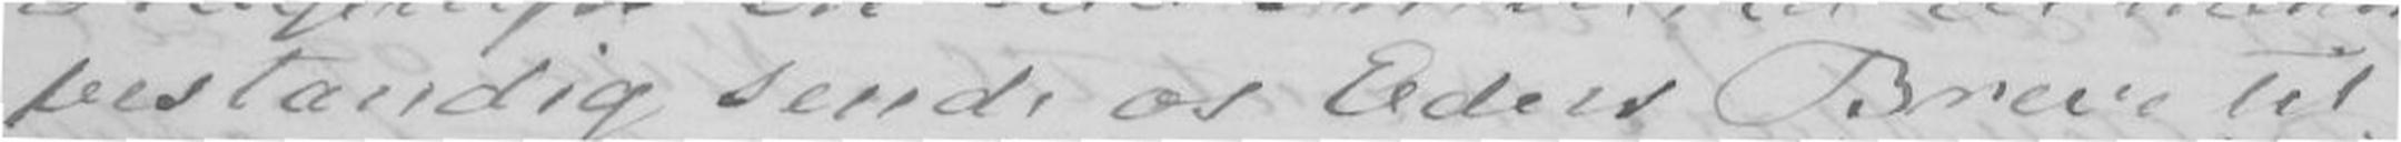bestandig sende os Eders Breve til
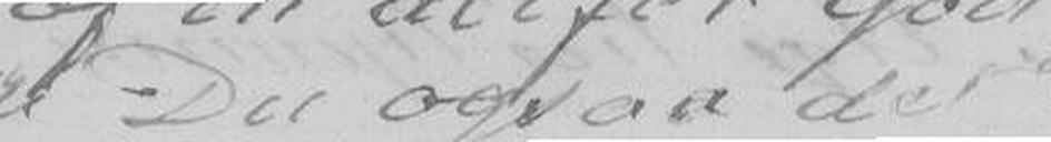Du ogsaa det?
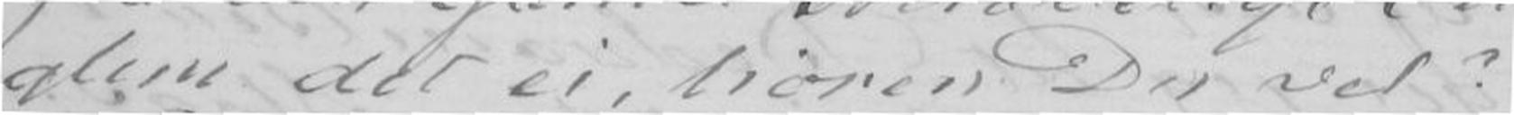glem det ei, hører Du vel?
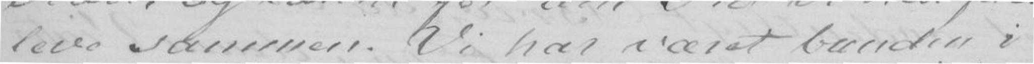leve sammen. Vi har været bunden i
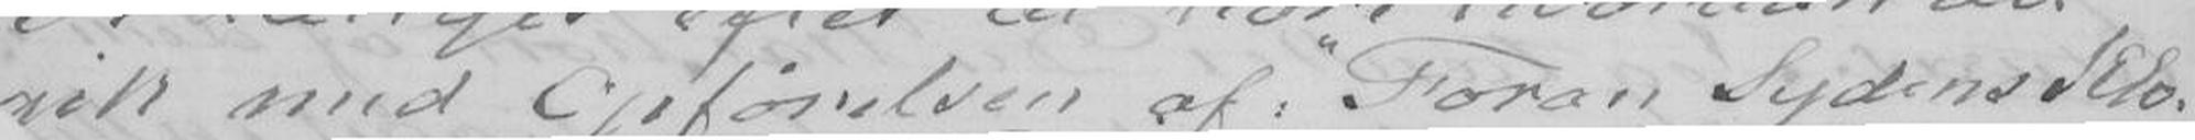gik med Opførelsen af: "Foran Sydens Klo-
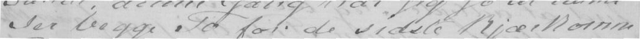Jer begge To for sidste kjærkomne
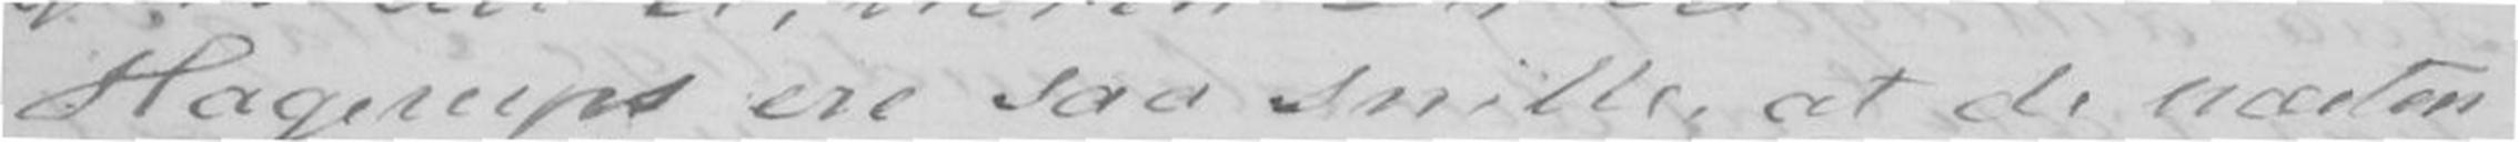Hagerups ere saa snille, at de næsten
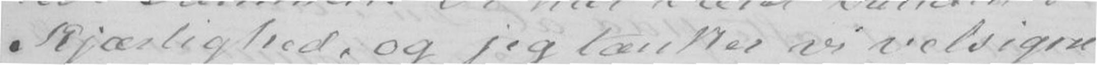kjærlighed, og jeg tænker vi velsigne
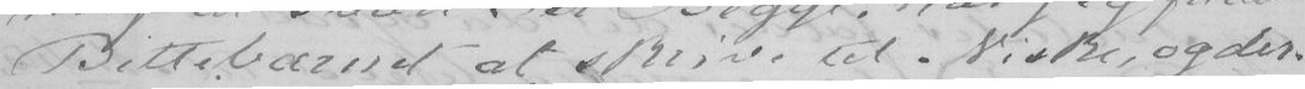Bettebarnet at skrive til Niske, og der-
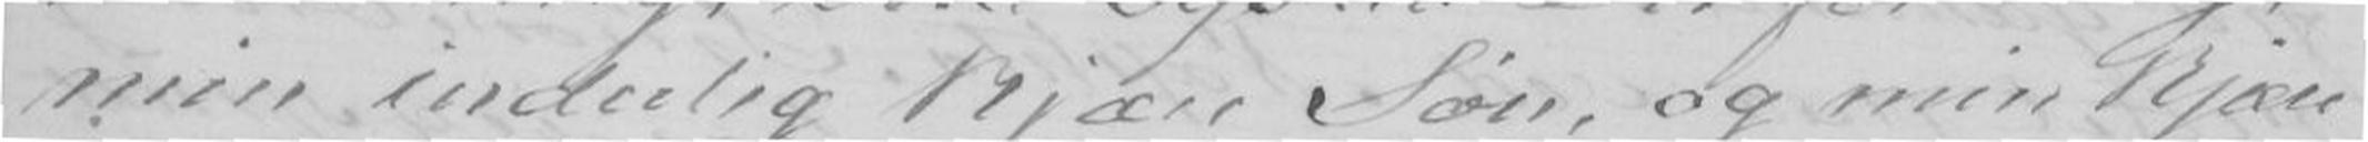min inderlig kjære Søn, og min kjære
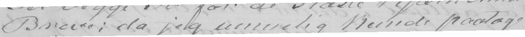Breve; da jeg umuelig kunde paatage
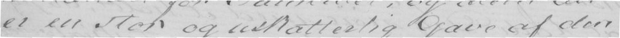er en stor og uskatterlig Gave af den
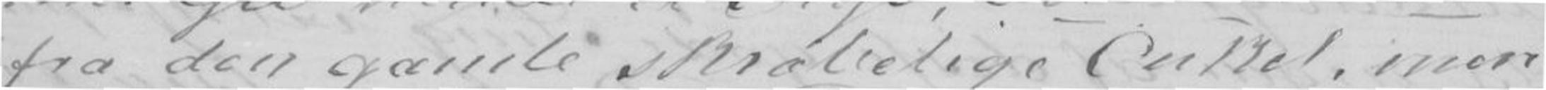fra den gamle skrøbelige Onkel, men
